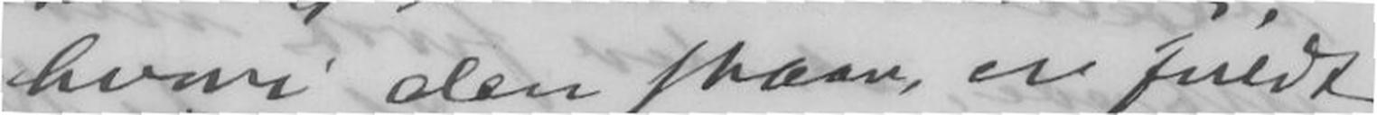hvori den staar, er fuldt
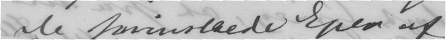De forinskede Eplr af
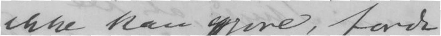ikke kan gjøre, fordi
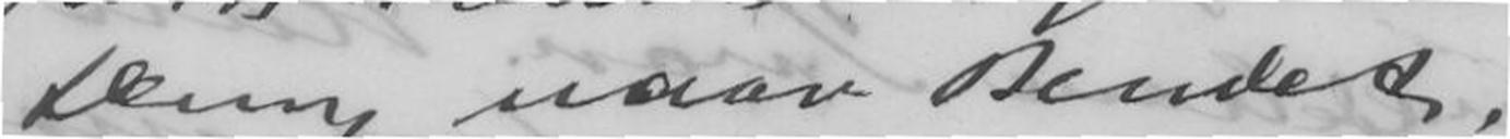Dem naar Bindet
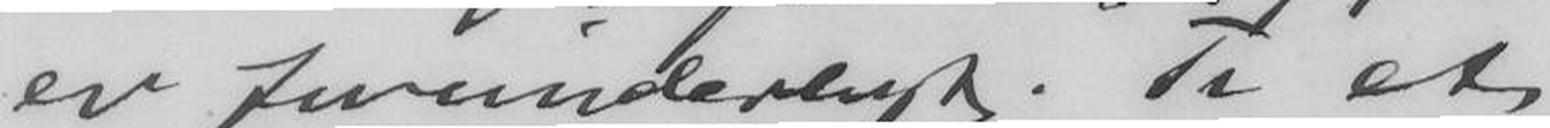er forunderligt. Ti et
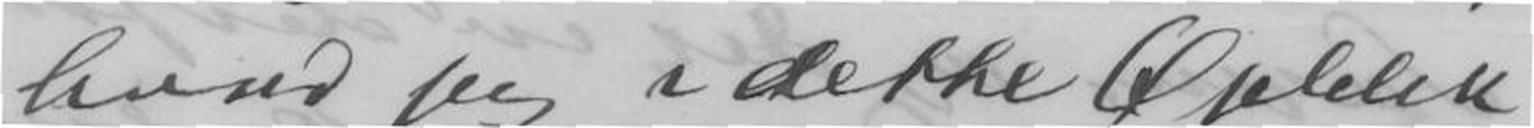hvad jeg i dette Øjeblik
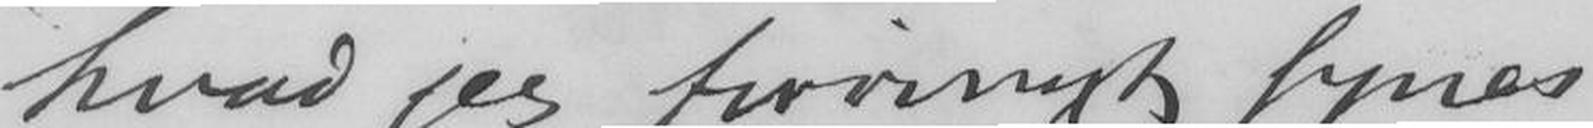hvad jeg forøvgigt synes
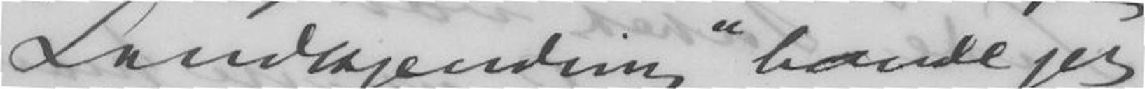Landkjending havde jeg
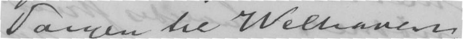Sangen til Welhaven,
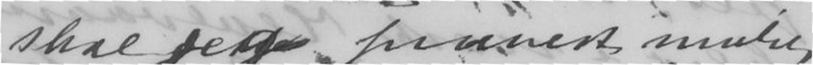skal jeg snarest mulig
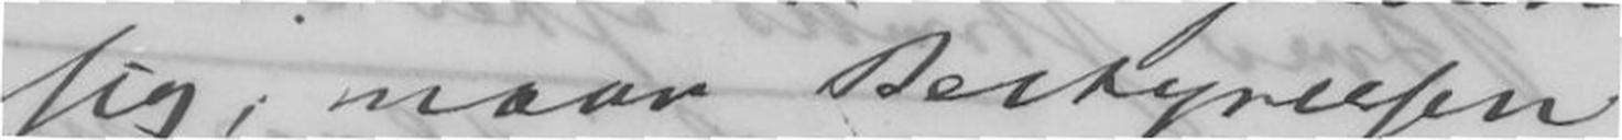sig, naar Bestyrelsen
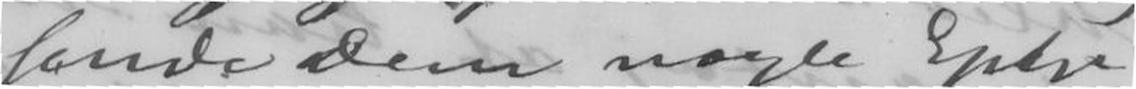sænde dem nogle Eplr
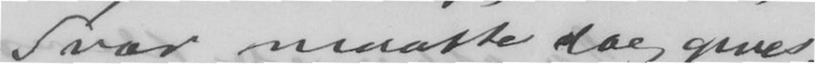Svar maatte dog gives,
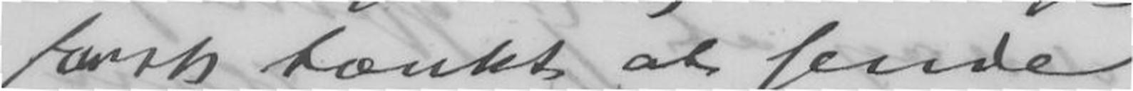først tænkt at sende
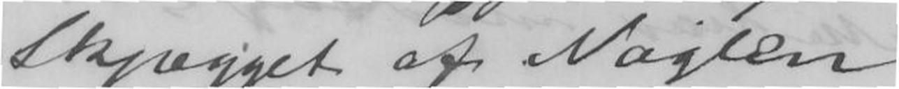skjægget af Nøglen
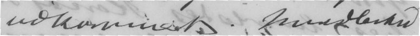udkommet. Imidlertid
